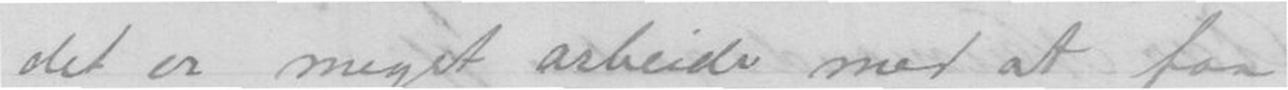det er meget arbeide med at faa
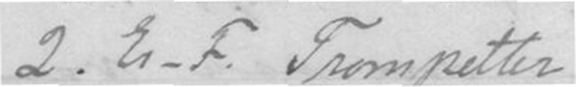2. Es-F Trompetter
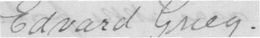Edvard Grieg.
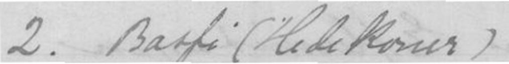2. Basfi (Hedekoner)
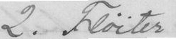2. Fløyter
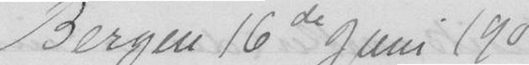Bergen 16de Juni 1901
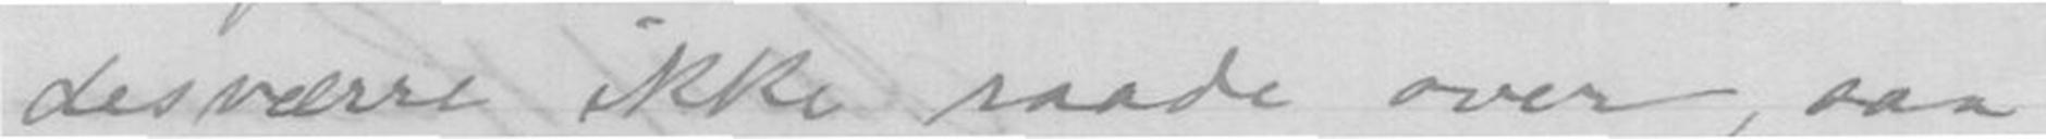desværre ikke raade over, saa
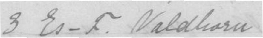3. Es-F Valdhorn
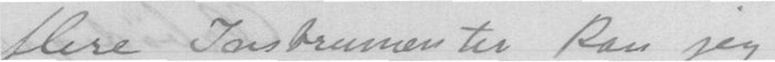flere instrumenter kan jeg
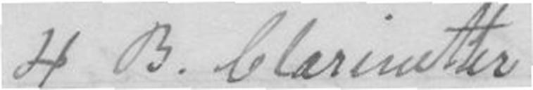4. B.Clarinetter
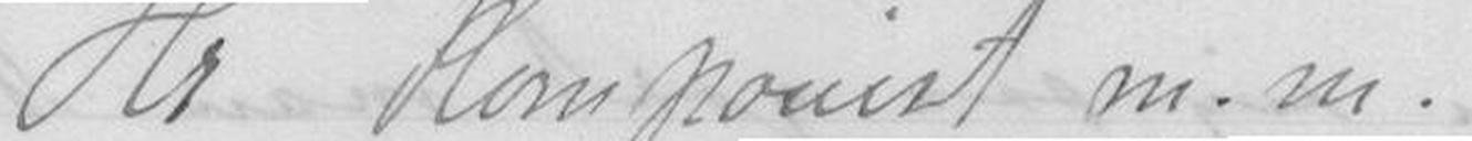Hr. Komponist m.m.
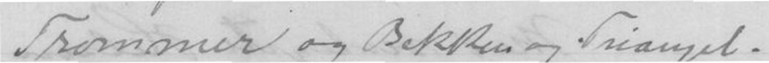Trommer og Bekken og Triangel.
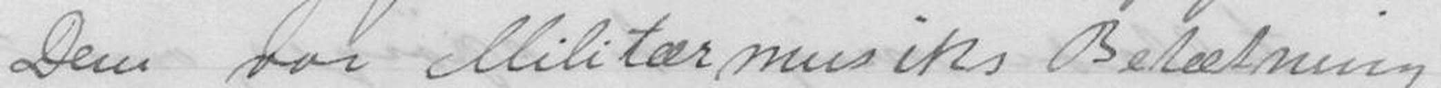Dem vor Militærmusiks Besætning
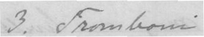3. Tromboni
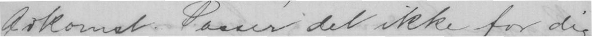Adkomst. Passer det ikke for dig
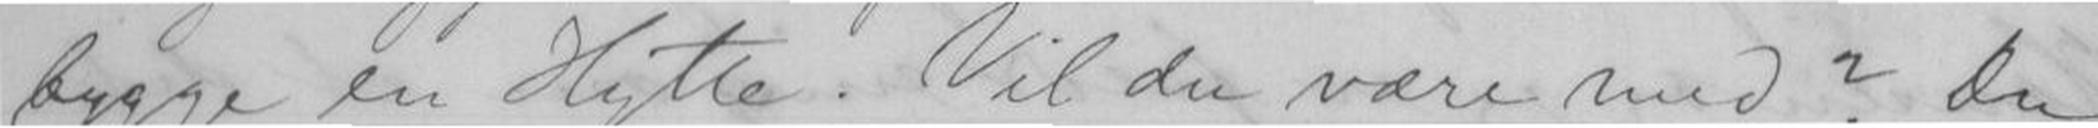bygge en Hytte. Vil du være med? Du
